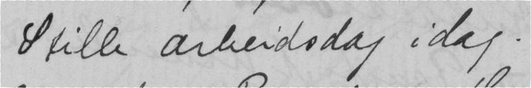Stille arbeidsdag idag.
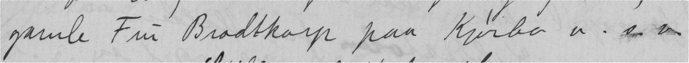gamle Fru Brodtkorp paa Kjørbo o.s.v.
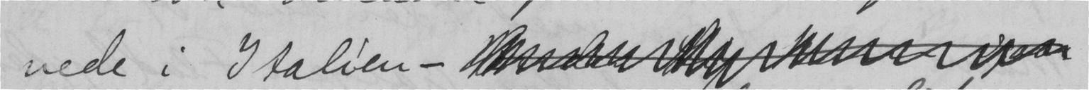nede i Italien -
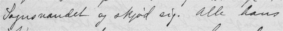Sognsvandet og skjød sig. Alle hans
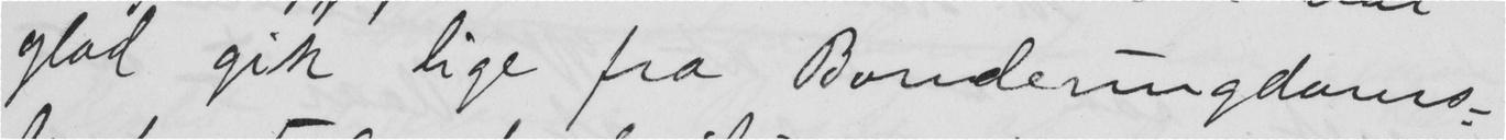glad gik lige fra Bondeungdoms-
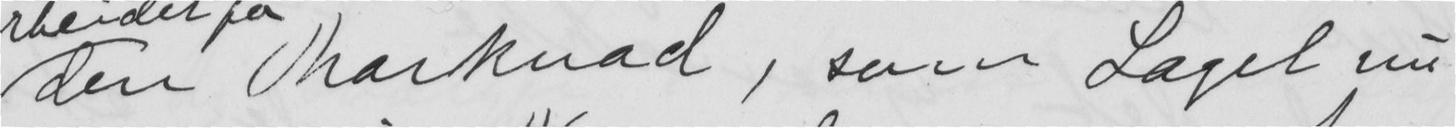den Marknad, som Laget nu
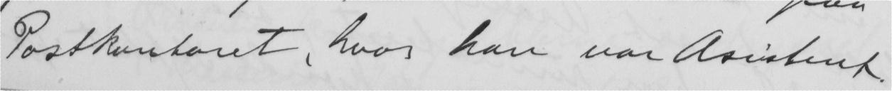Postkontoret, hvor han var Asistent.
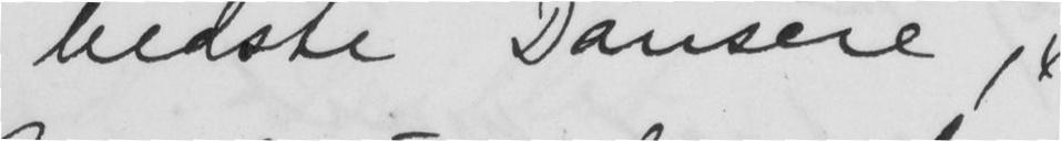bedste Dansere,
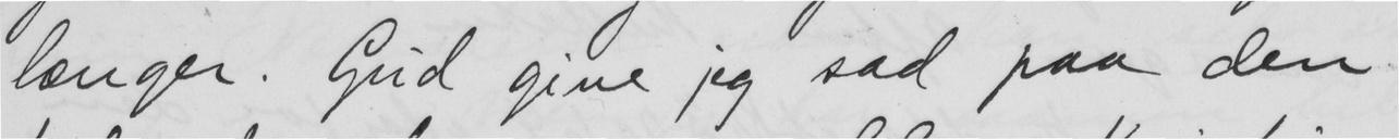længer. Gud give jeg sad paa den
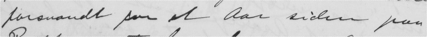forsvandt for et Aar siden paa
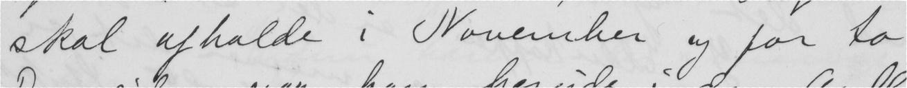skal afholde i November og for to
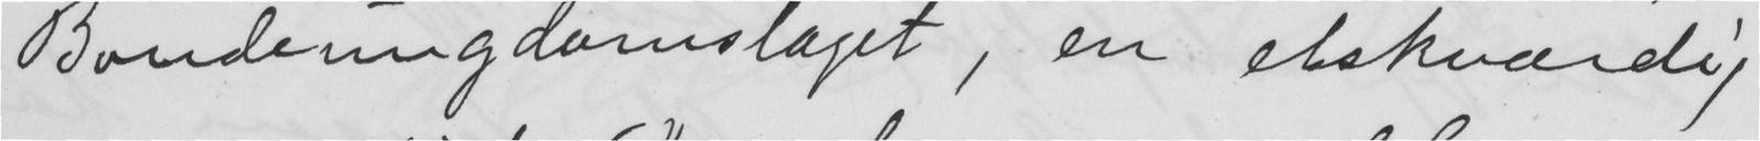Bondeungdomslaget, en elskværdig
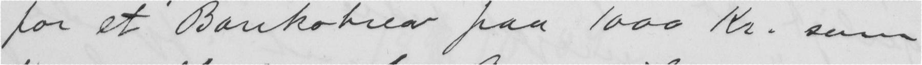for et Bankobrev paa 1000 Kr. som
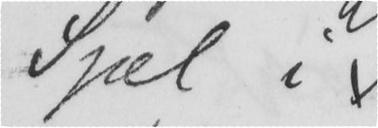sjæl i
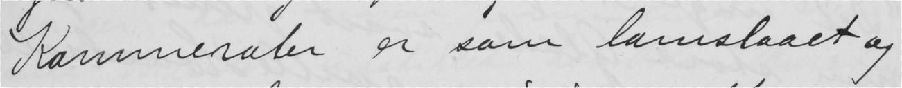Kammerater er som lamslaaet og
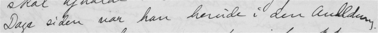Dage siden var han herude i den Anledning.
